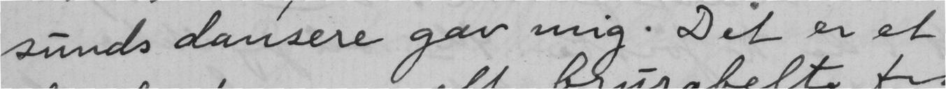sunds dansere gav mig. Det er et
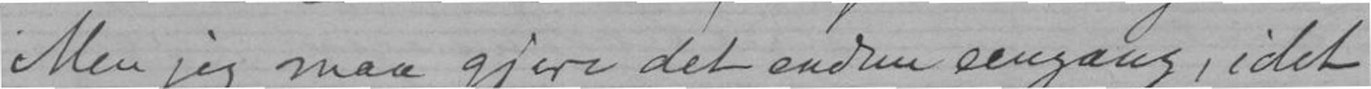Men jeg maa gjere det endnu eengang. idet
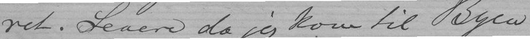ret. Senere da jeg kom til Byen
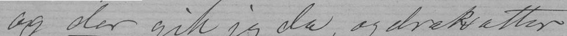og der gik jeg da, og drak atter
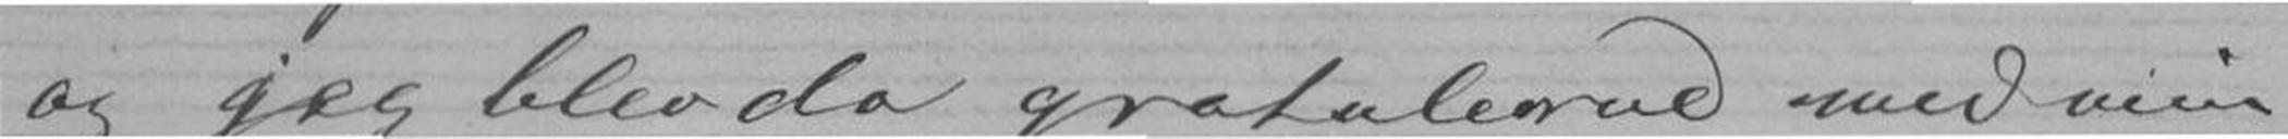og jeg blev da gratulered med min
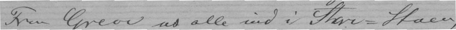Fru Greve os alle ind i Stor-Stuen,
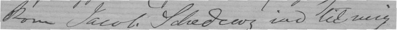kom Jakob Schediwy ind til mig
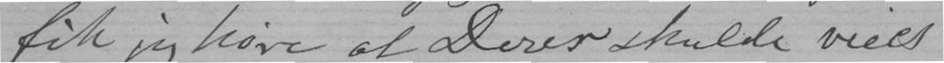fik jeg høre at Deres skulde vies
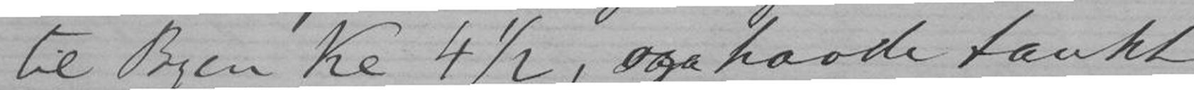til Byen Kl 4 1/2, og havde tænkt
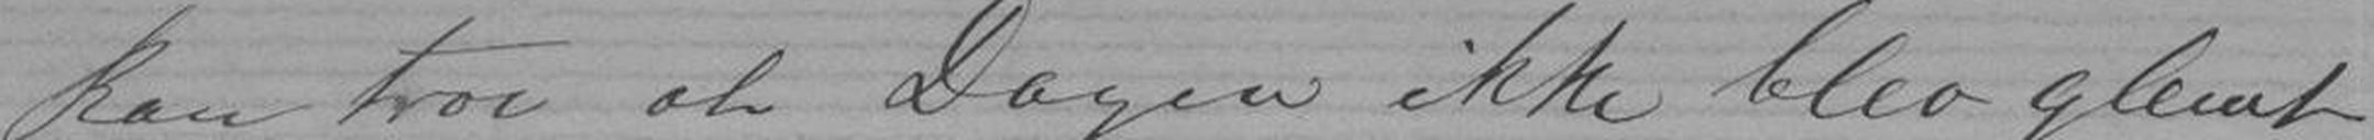kan tro at Dagen ikke blev glemt.
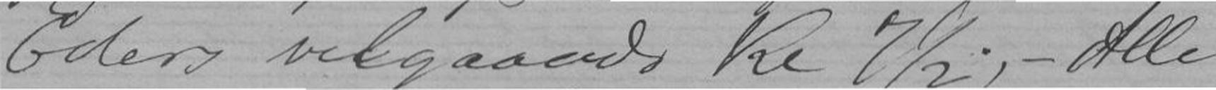Eders velgaaende Kl 7 1/2, - Alle
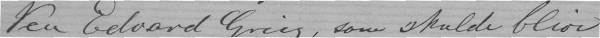Ven Edvard Grieg, som skulde blive
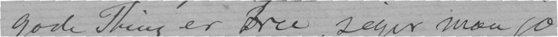gode Thing er tree, siger man jo
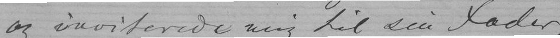og inviterede mig til sin Fader
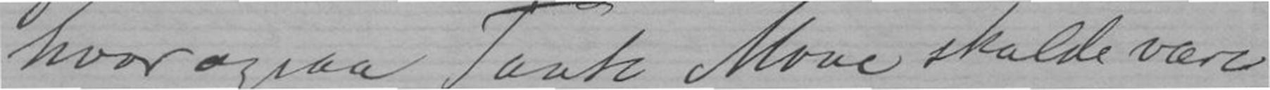hvor ogsaa Tante Mone skulde være
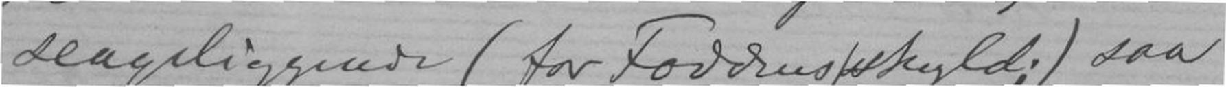sengeliggende (for Foddens/skyld;) saa
